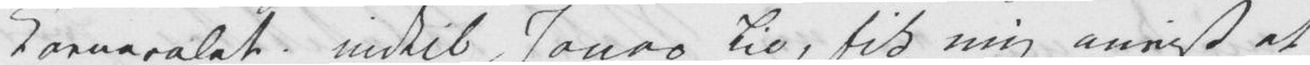Karnevalet – indtil Jonas Lie, fik mig anvist et
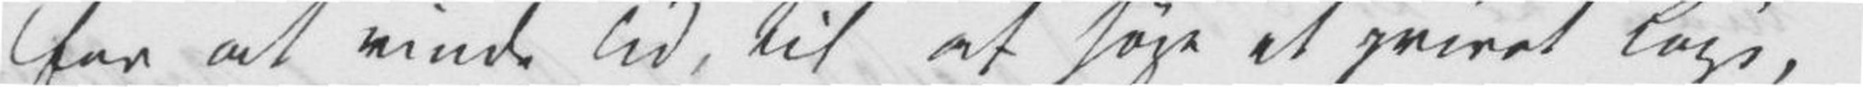for at vinde Tid, til at søge et privat Logi,
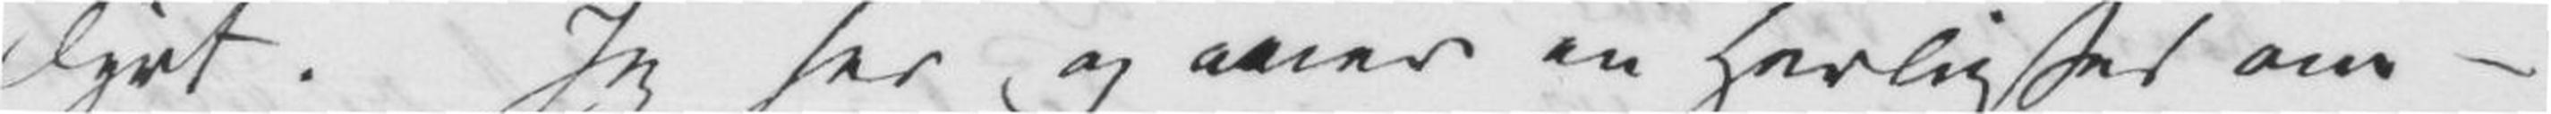dyrt. Jeg ser og aner en Herlighed om¬
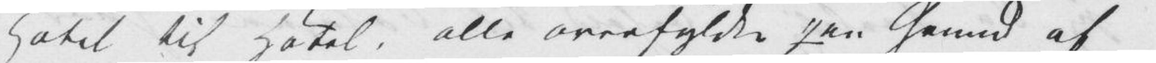Hotel til Hotel, alle overfyldte paa Grund af
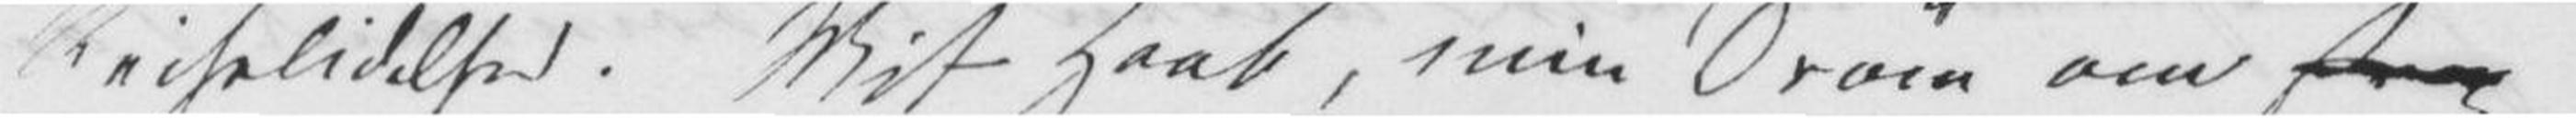Reiselidelser. Mit Haab, min Drøm om straks
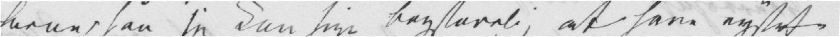leren, saa jeg kan sige bogstavelig at have rystet
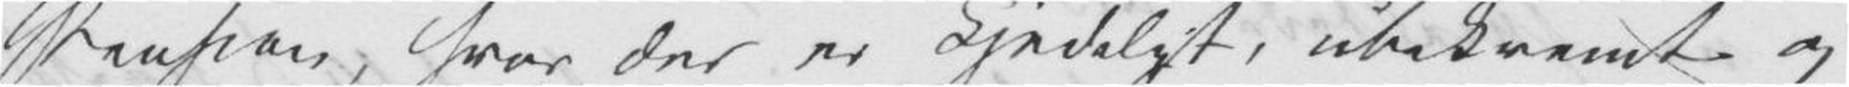Pension, hvor der er kjedeligt, ubekvemt og
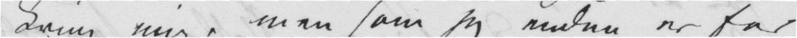kring mig, men som jeg endnu er for
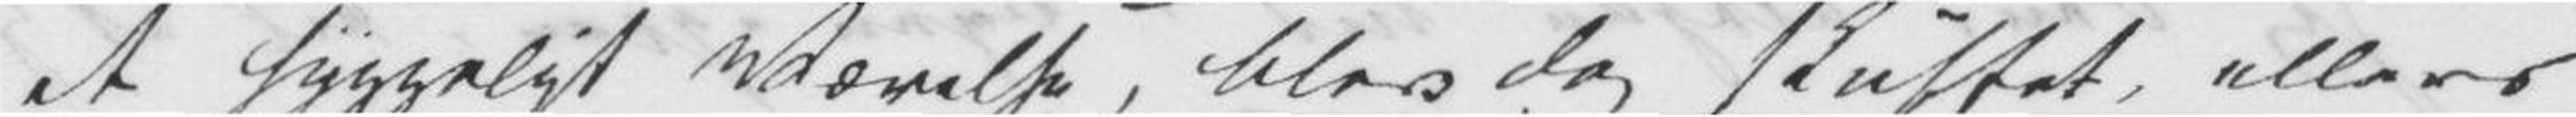i et hyggeligt Værelse, blev dog skuffet, ellers
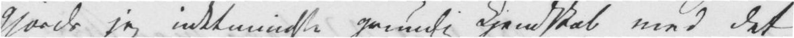gjorde jeg idetmindste grundig Kjendskab med det
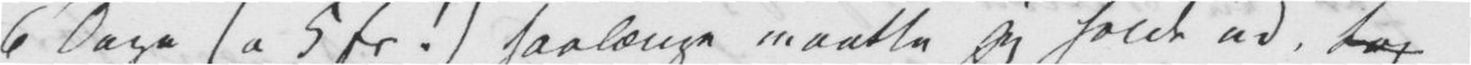6 Dage (a 5 fr!) saalænge maatte jeg holde ud, tog
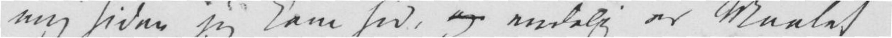mig siden jeg kom hid, endelig er Maalet
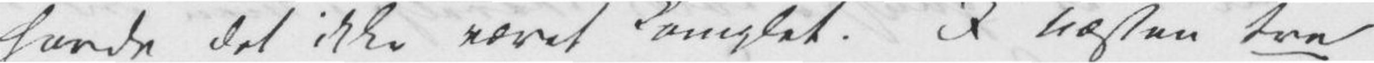havde det ikke været komplet. I næsten tre
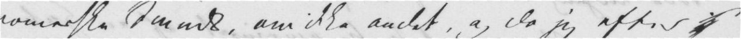romerske Smuds, om ikke andet, og da jeg efter
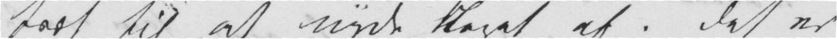træt til at nyde Noget af. Det er
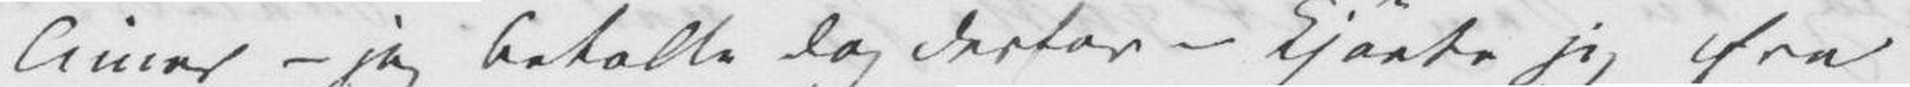Timer – jeg betalte dog derfor – kjørte jeg fra
